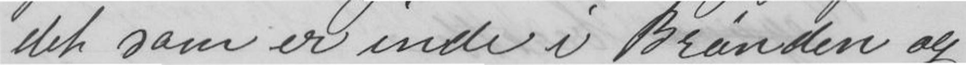det som er inde i Brønden og
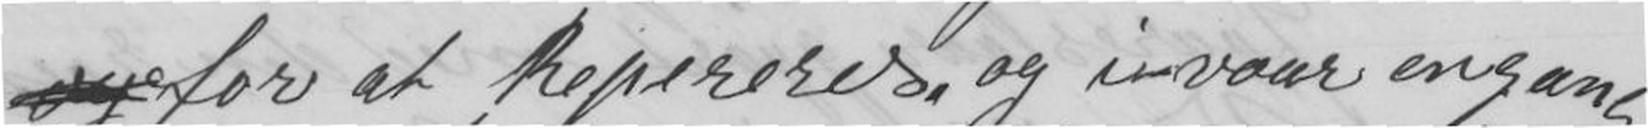for at Repereres, og ivaar engang
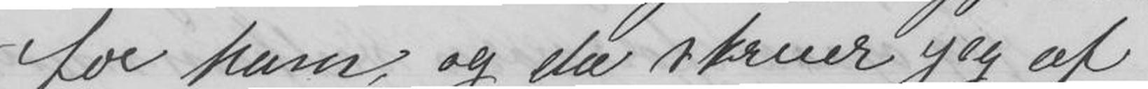- for ham og da skruer jeg af
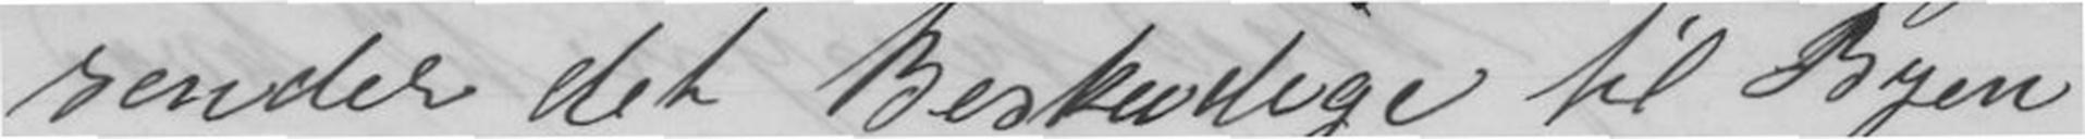sender det Beskudige til Byen
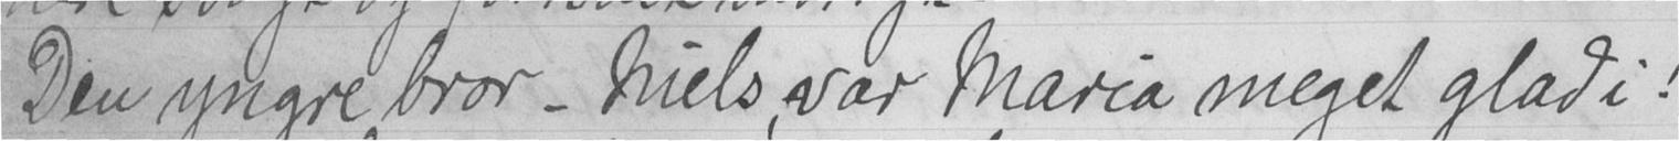Den yngre bror - Niels, var Maria meget glad i!
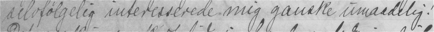selvfølgelig interesserede mig ganske umaadelig!
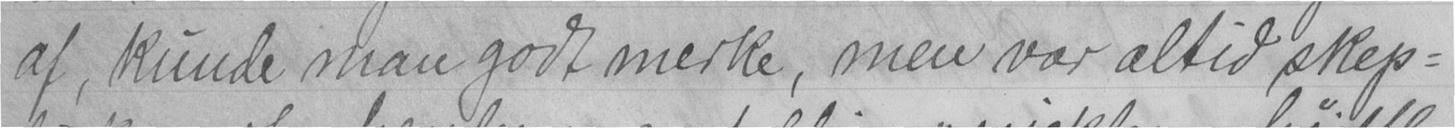af, kunde man godt merke, men var altid skep-
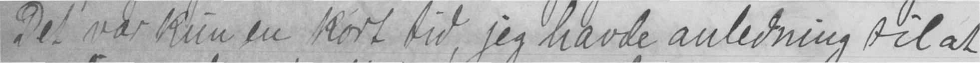Det var kun en kort tid, jeg havde anledning til at
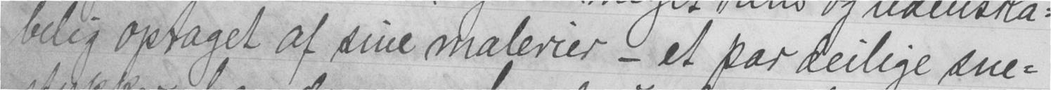belig optaget af sine malerier - et par deilige sne-
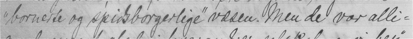"bornerte og spidsborgerlige" væsen. Men de var alli-
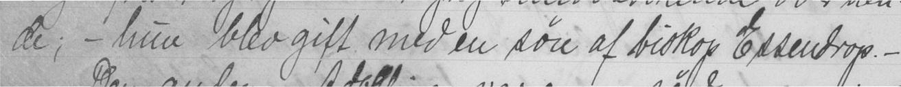de; - hun blev gift med en søn af biskop Essendrop. -
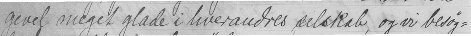gevel meget glade i hverandres selskab, og vi besøg-
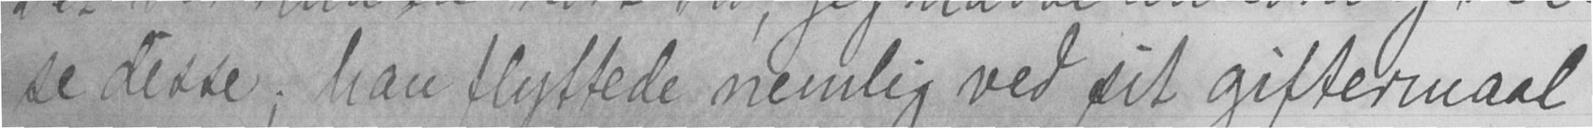se disse; han flyttede nemlig ved sit giftermaal
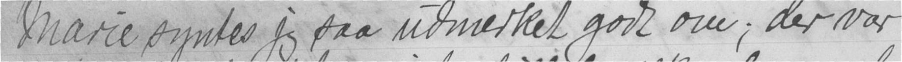Marie syntes jeg saa udmerket godt om; der var
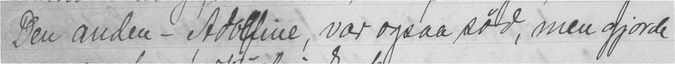Den anden - Adolfine, var ogsaa sød, men gjorde
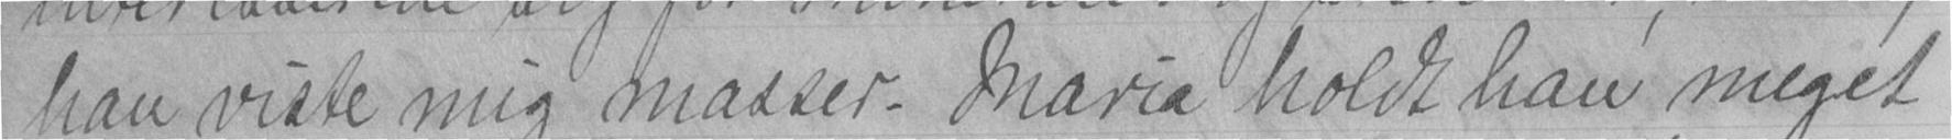han viste mig masser. Maria holdt han meget
